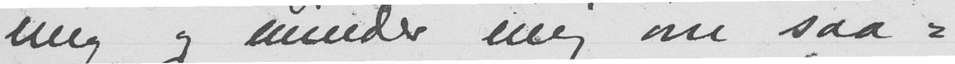mig og minder mig om saa-
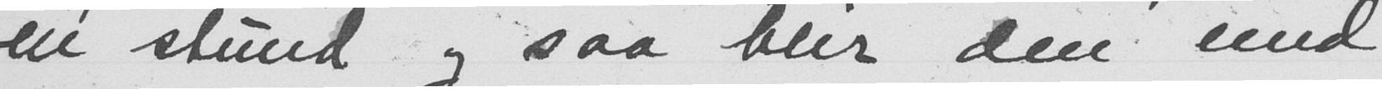en stund og saa blir den med
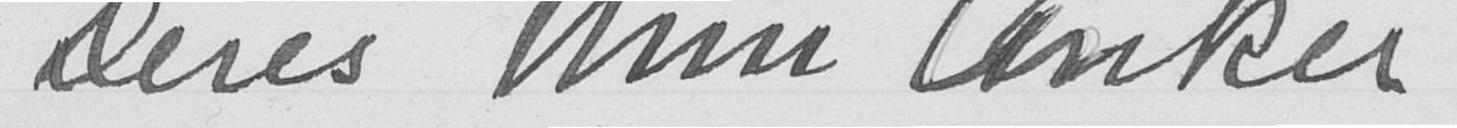deres Nini Anker
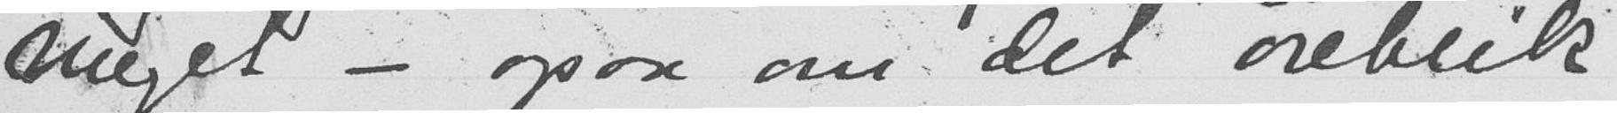meget - ogsaa om det øieblik
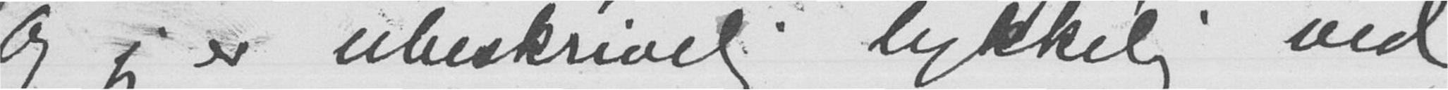Og jeg er ubeskrivelig lykkelig ved
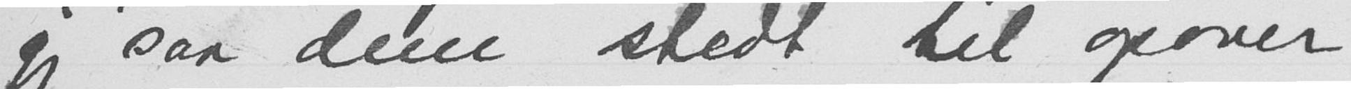jeg saa dem stedt til opover
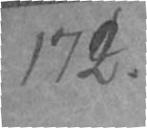172.
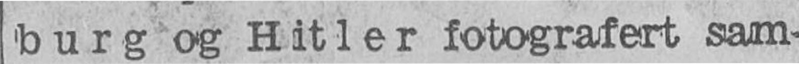burg og Hitler fotografert sam-
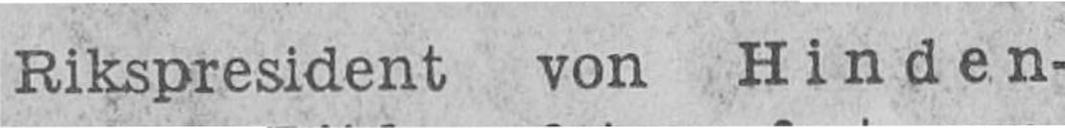Rikspresiden von Hinden-
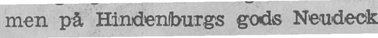men på Hindenburgs gods Neudeck
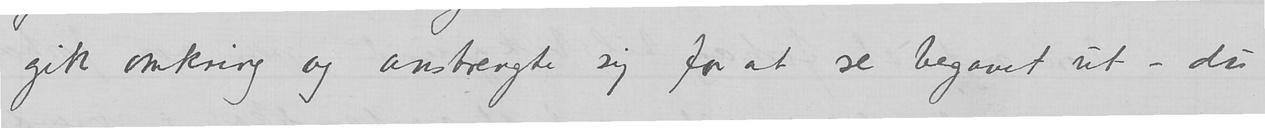gik omkring og anstrengte sig for at se begavet ut – du
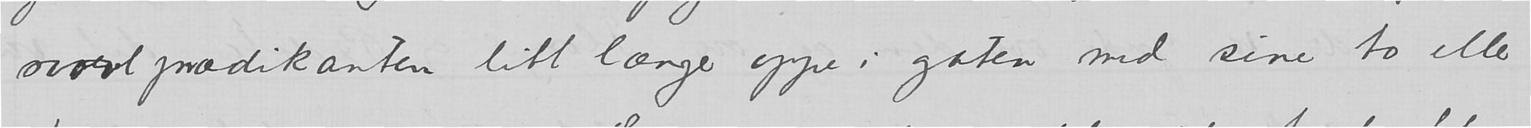tilhørere og svovlprædikanten litt længer oppe i gaten med sine to
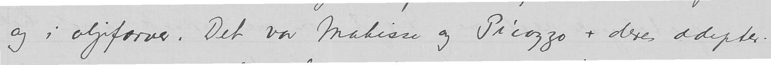og i oljefarver. Det var Matisse og Picazzo + deres adepter.
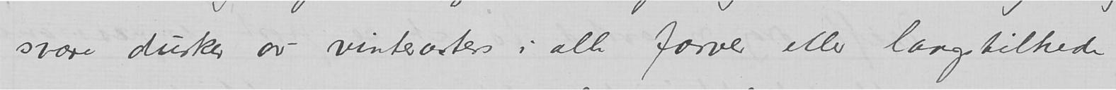svære dusker av vinterasters i alle farver eller langstilkede
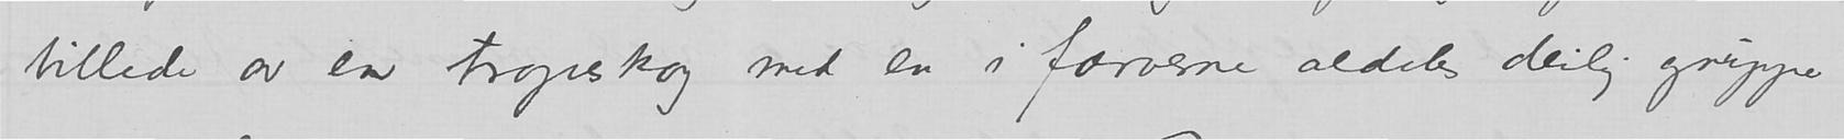billede av en tropeskog med en i farverne aldeles deilig gruppe
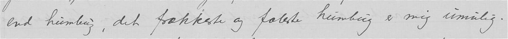end humbug, det frækkeste og fæleste humbug er mig umulig.
